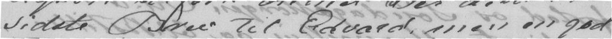sidste Brev til Edvard, men en god
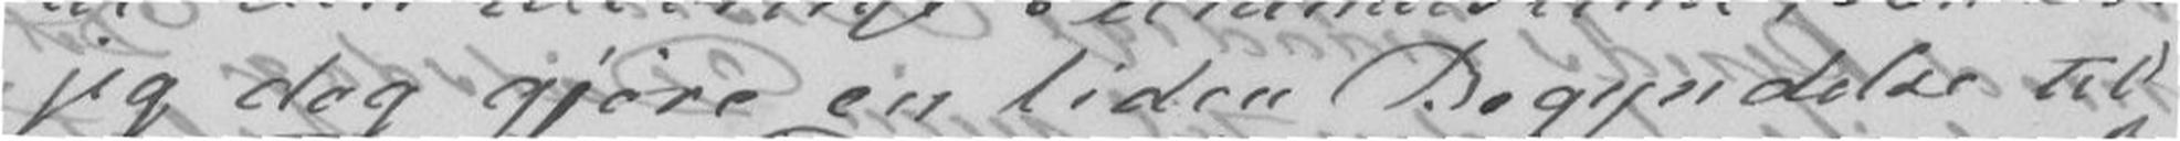jeg dog gjøre en liden Begyndelse til
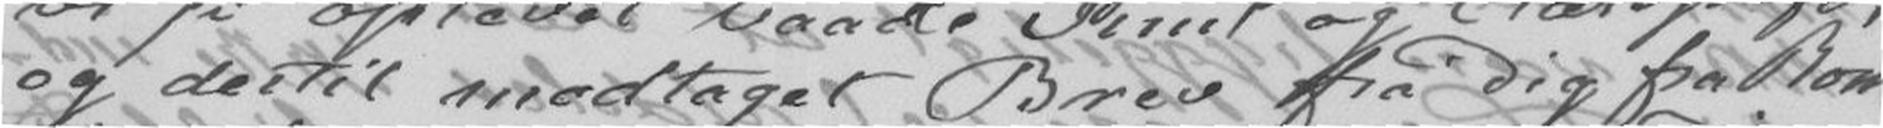og dertil modtaget Brev fra Dig fra Rom.
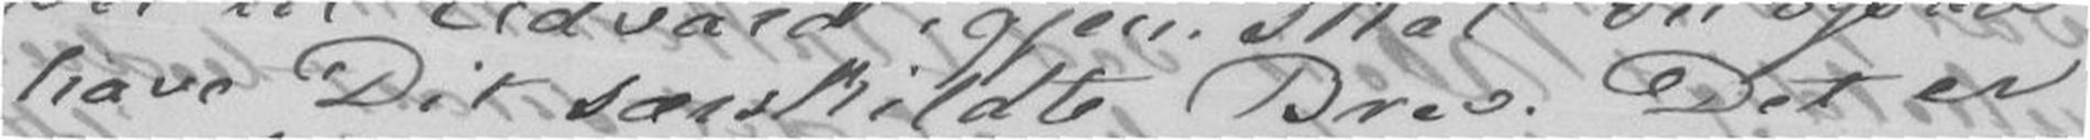have Dit særskilte Brev. Det er
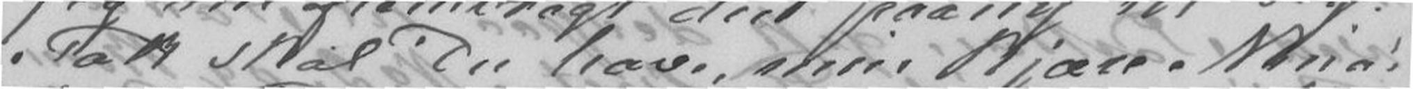Tak skal Du have, min kjære Nina!
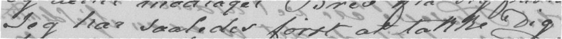Jeg har saaledes først at takke Dig
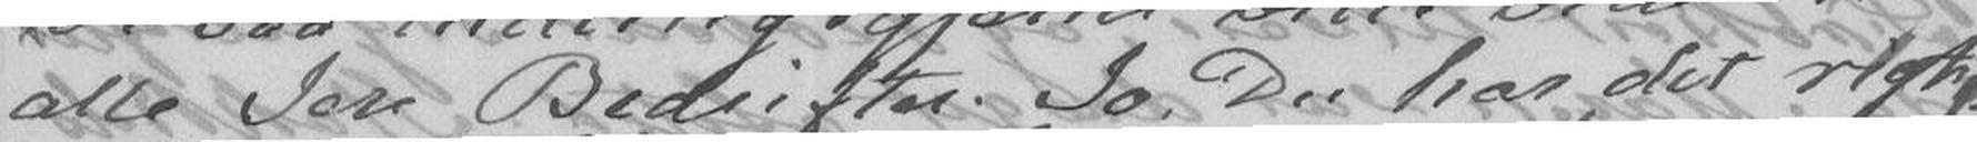alle Jer Bedrifter. Jo, Du har det rigtig-
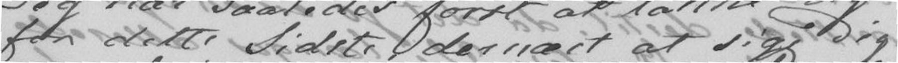for dette Sidste, dernæst at sige Dig
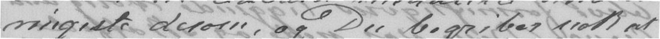ringeste derom, og Du begriber nok at
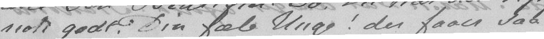nok godt. Din Fæle Unge! der faaer saa
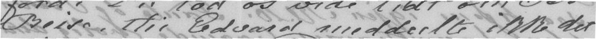Reise, thi Edvard meddelte ikke det
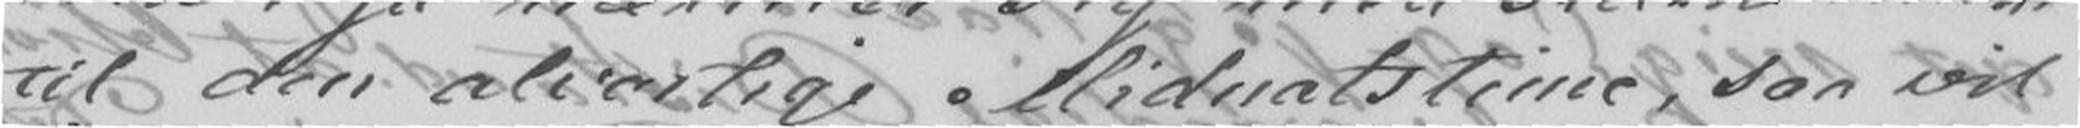til den alvorlige Midnatstime, saa vil
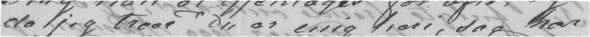da jeg troer Du er enig heri, saa har
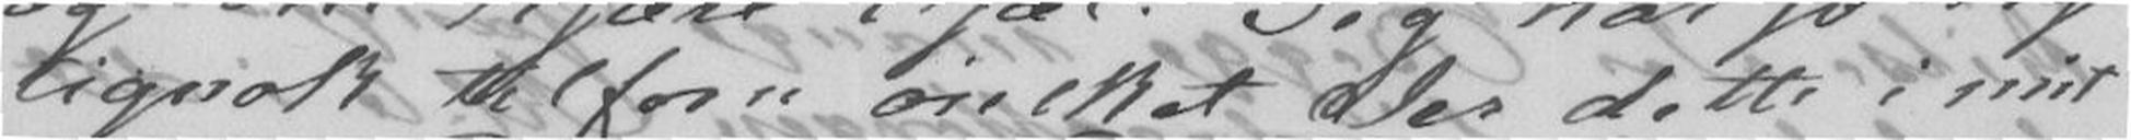tignok tilforn ønsket Jer dette i mit
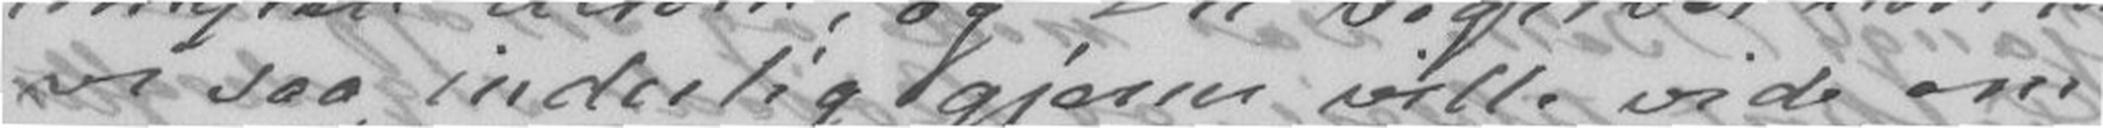vi saa inderlig gjerne ville vide om
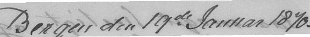Bergen den 19de Januar 1870.
